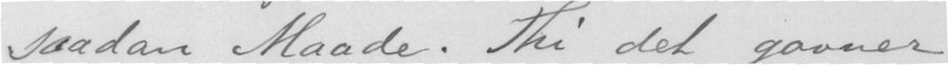saadan Maade. Thi det gavner
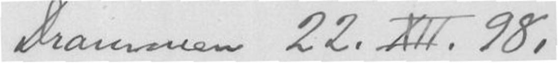Drammen 22.XII.98.
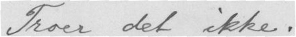Troer det ikke.
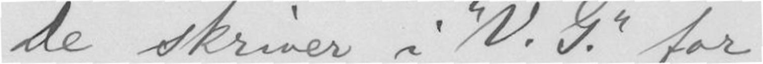De skriver i "V.G." for
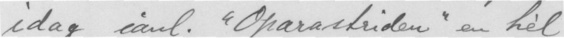idag ianl. "Oparastriden" en hél
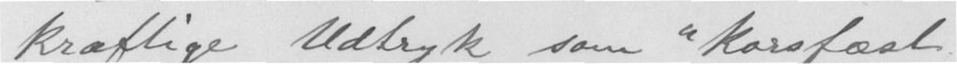kraftige Udtryk som "Korsfæst
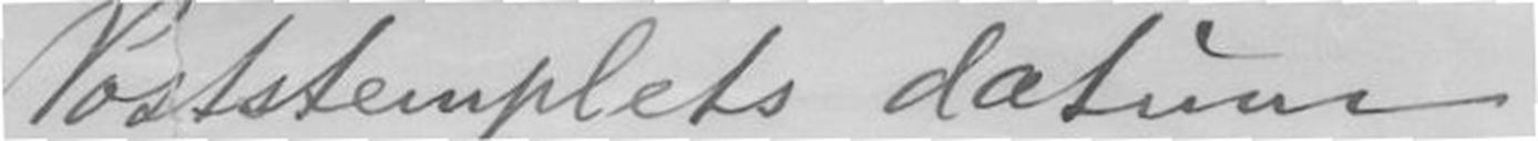Poststemplets datum.
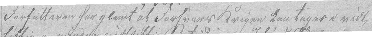Forfatteren har glemt at Forsvarskrigen kan tages i vidt-
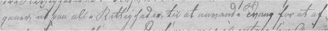gaaer ud paa alle Rettigheder til at anvende Tvang for at af-
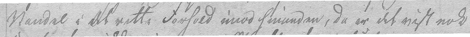Vandel i det rette Forhold imod hinanden, da er det vist nok
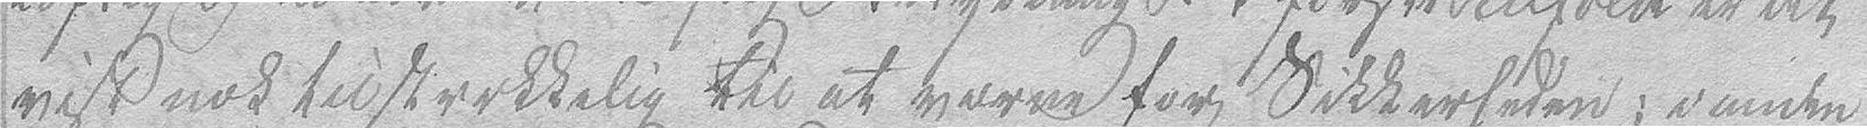vist nok tilstrækkelig til at værne for Sikkerheden; i anden
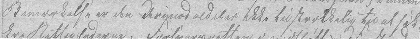Bemærkelse er den derimod aldeles ikke tilstrækkelig til at sik-
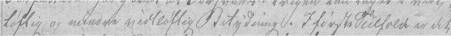løftig og mindre vidtløftig Betydning. I første Tilfælde er det
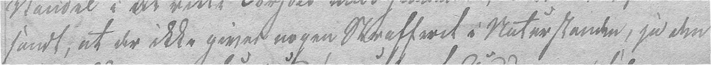sandt, at der ikke gives nogen Strafferet i Naturstanden, ja den
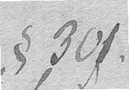§ 301.
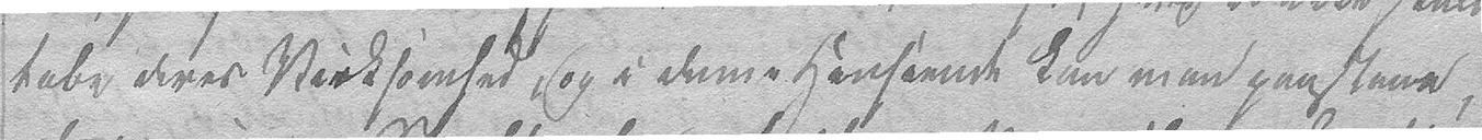tabe deres Virksomed, og i denne Henseende kan man paastaae,
